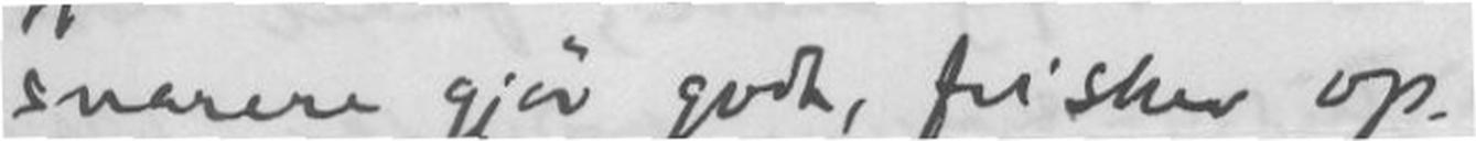snarere gjør godt, frisker op.
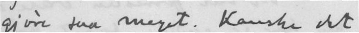gjøre saa meget. Kanske det
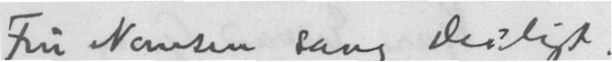Fru Nansen sang deiligt.
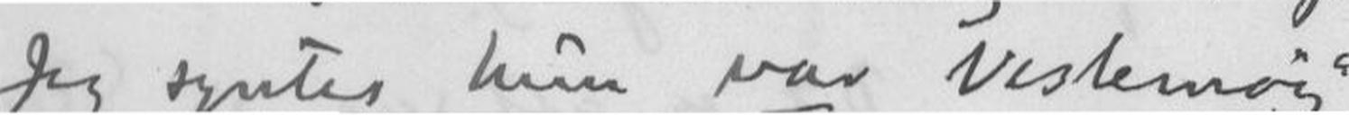Jeg syntes hun var Veslemøy,
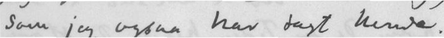som jeg ogsaa har sagt hende.
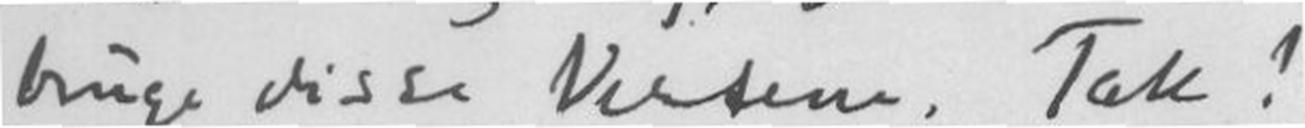bruge disse Versene. Tak!
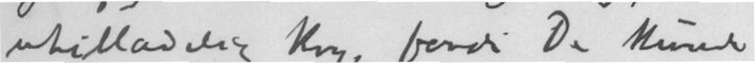utilladelig kry, fordi De kunde
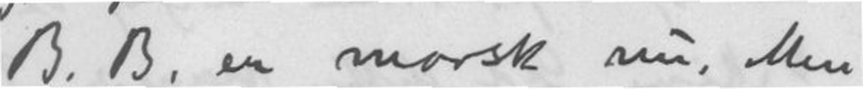B. B. er morsk nu. Men
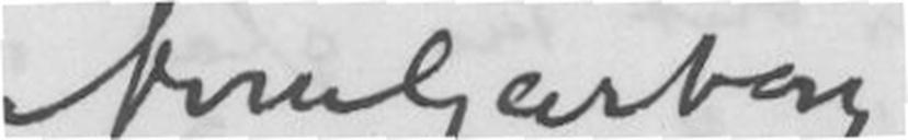Arne Garborg
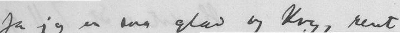Ja jeg er saa glad og kry, rent
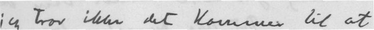jeg tror ikke det kommer til at
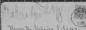Frankfurt a. M.)
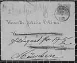(bei
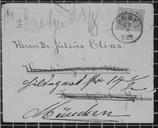Homburg v. d. Höhe.
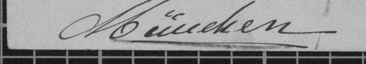Herrn Dr. Julius Elias.
